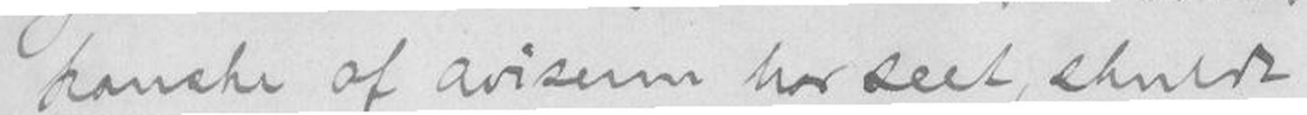kanske af avisene har seet, skulde
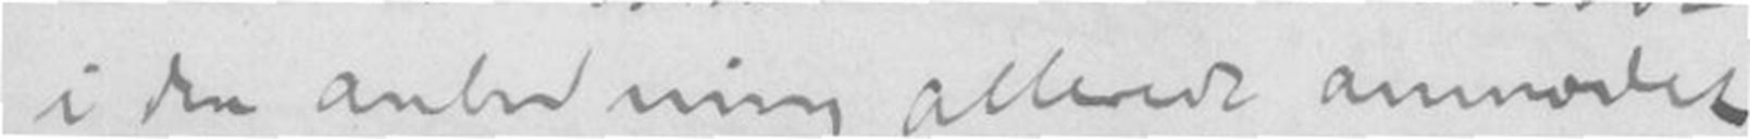i den anledning allrede anmodet
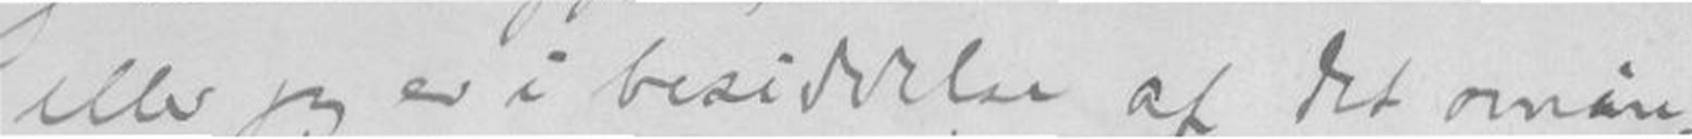eller jeg er i besiddelse af det omin
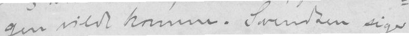gen vilde komme. Svendsen siger
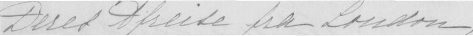Deres Afreise fra London,
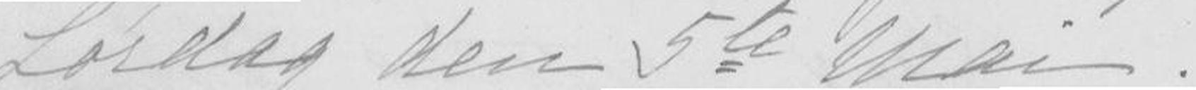Lørdag den 5te Mai.
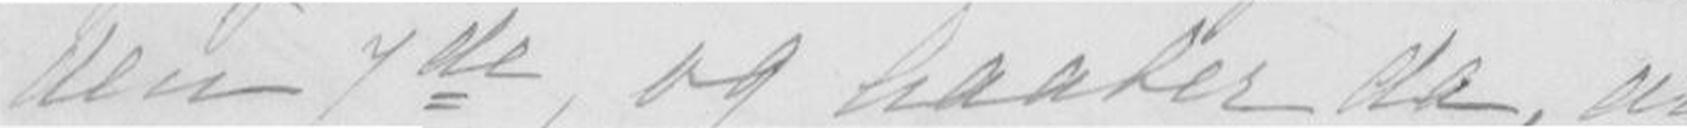den 7de, og haaber da, at
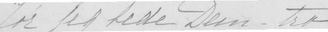Tør jeg bede Dem - tro
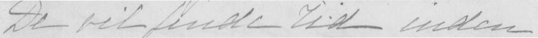De vil finde Tid inden
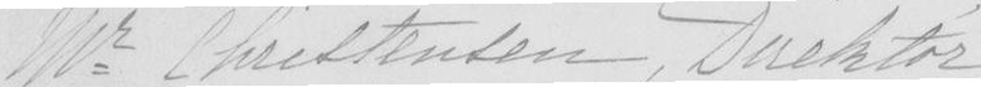Mr Christensen, Direktør
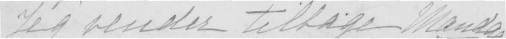Jeg vender tilbage Mandag
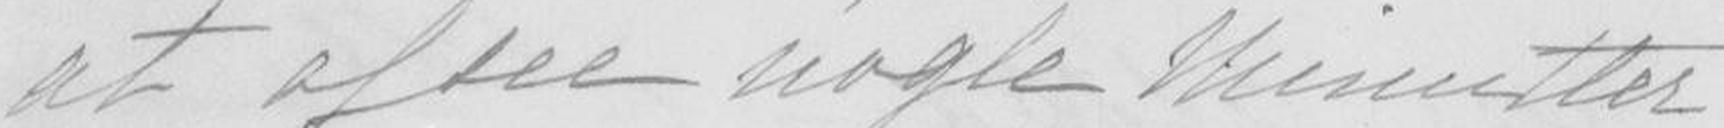at afsee nogle Minutter
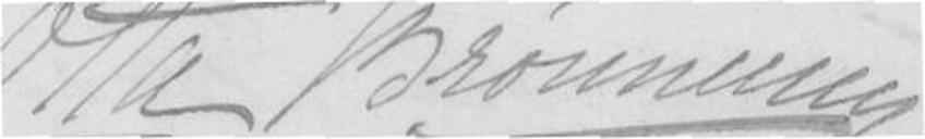Otta Brönnum
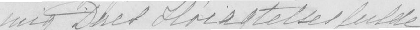mig Deres Høiagtelsesfulde
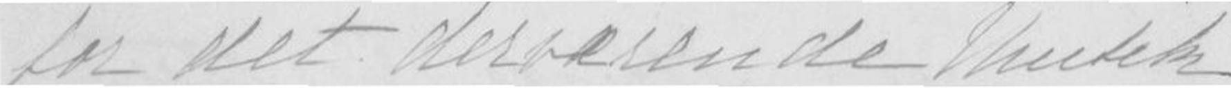for det derværende Musik
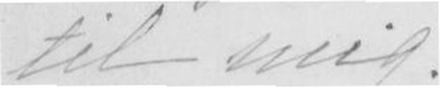til mig.
